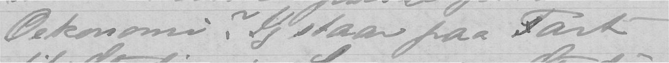Oekonomi? Jeg staar paa Tart
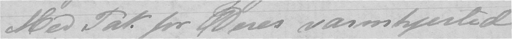Med Tak for Deres varmhjertede
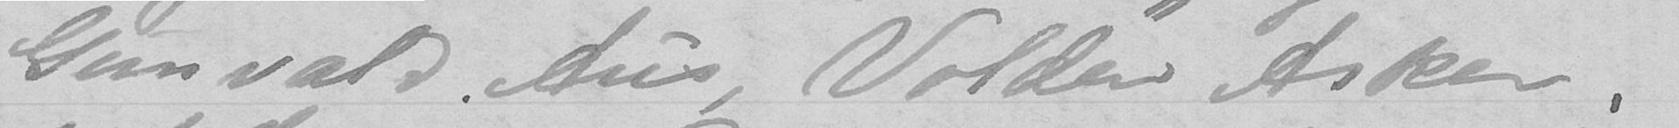Gunvald Aus, Volden Asker.
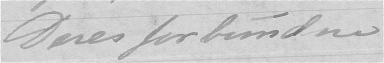Deres forbundne
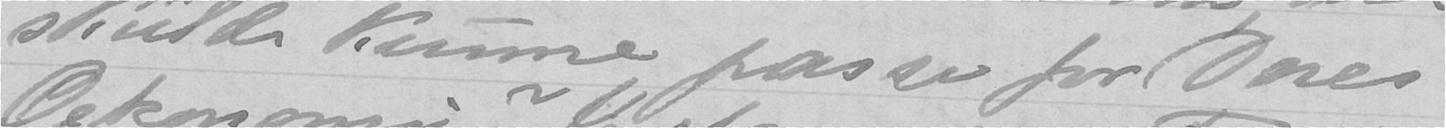skulde kunne passe for Deres
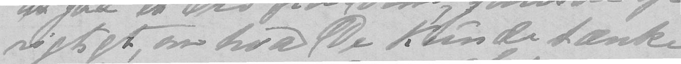rigtigt, om hvad De kunde tænke
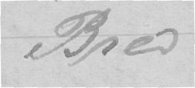Brev
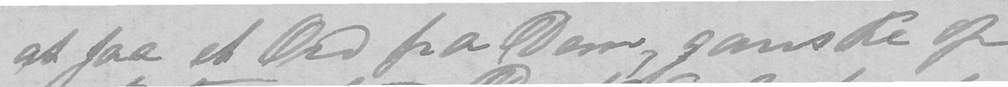at faa et Ord fra Dem, ganske op-
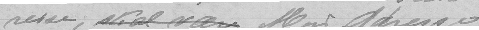reise, skal være Min Adresse
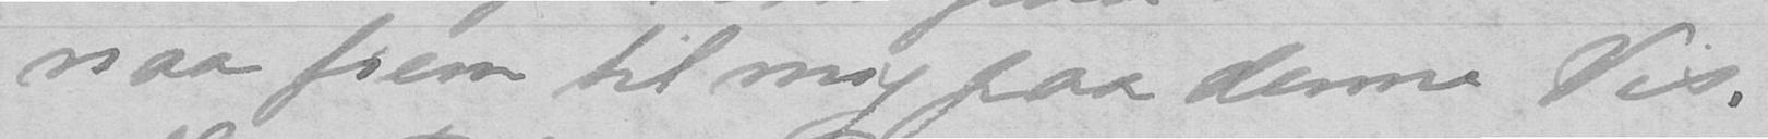naa frem til mig paa denne Vis.
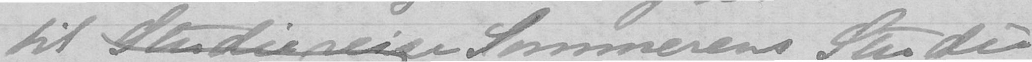til Studiereise Sommerens Studie-
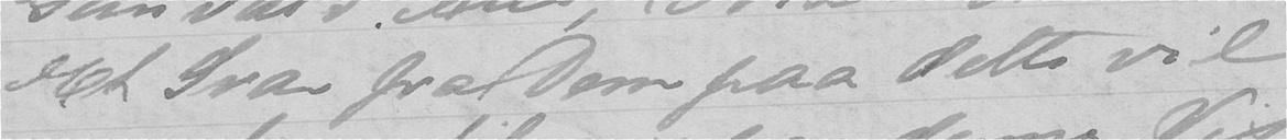Et Svar fra Dem paa dette vil
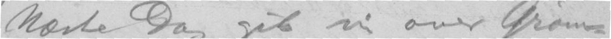Næste Dag gik vi over Grøm-
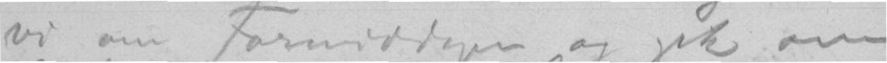vi om Formiddagen og gik om
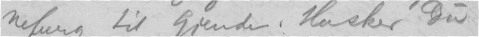neberg til Gjende. Husker Du,
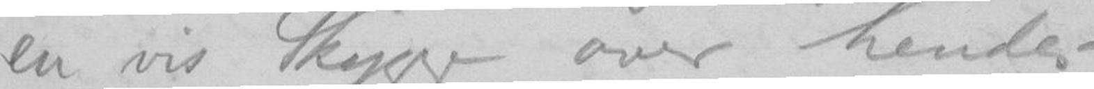en vis Skygge over hendes
